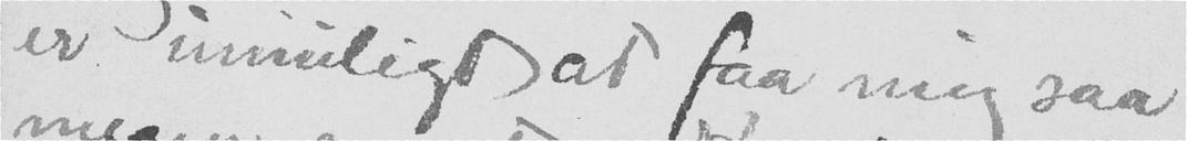er umuligt at faa mig saa
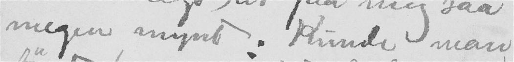megen mynt. Kunde man
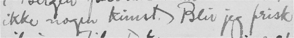ikke nogen kunst. Blir jeg frisk
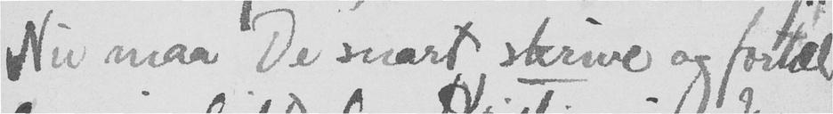Nu maa De snart skrive og fortæl
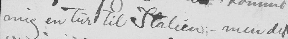mig en tur til Italien, - men det
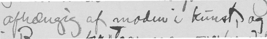afhængig af moden i kunst og
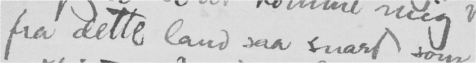fra dette land saa snart som
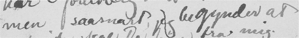men saasnart jeg begynder at
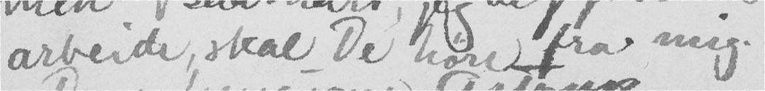arbeide, skal De høre fra mig.
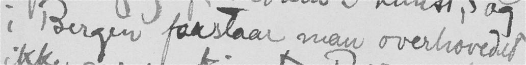i Bergen forstaar man overhovedet
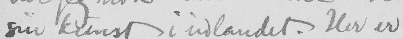sin kunst i udlandet. Her er
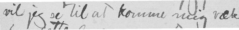vil jeg se til at komme mig væk
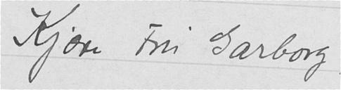Kjære Fru Garborg
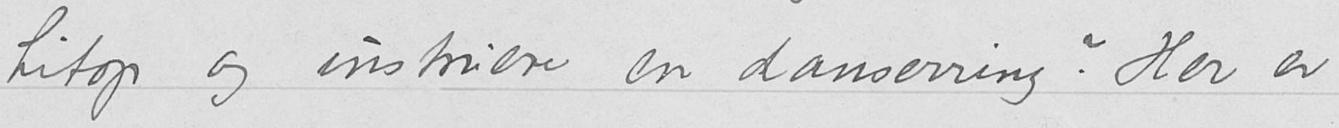hitop og instruere en danserring? Her er
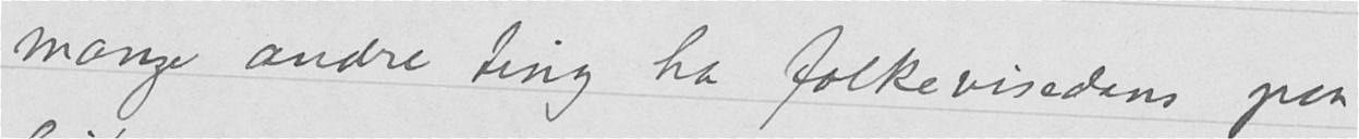mange andre ting ha folkevisedans paa
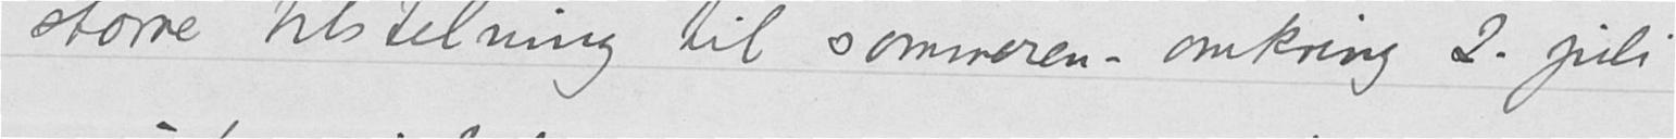større tilstelning til sommeren – omkring 2. juli
